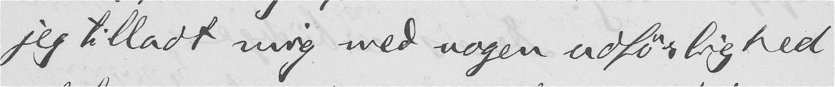jeg tilladt mig med nogen udførlighed
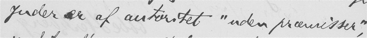ynder er af autoritet, "uden præmisser",
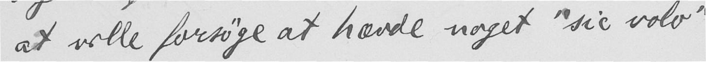at ville forsøge at hævde noget "sic volo".
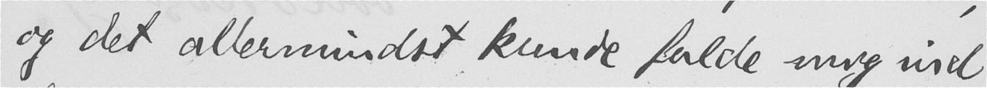og det allermindst kunde falde mig ind
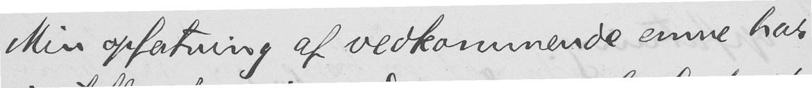Min opfatning af vedkommende emne har
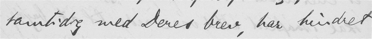samtidig med Deres brev, har hindret
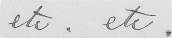etc. etc.
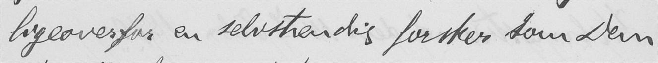ligeoverfor en selvstændig forsker som Dem
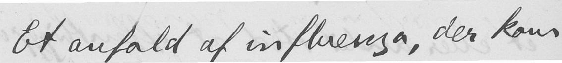Et anfald af influenza, der kom
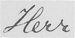Herr
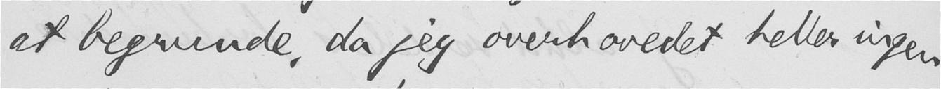at begrunde, da jeg overhovedet heller ingen
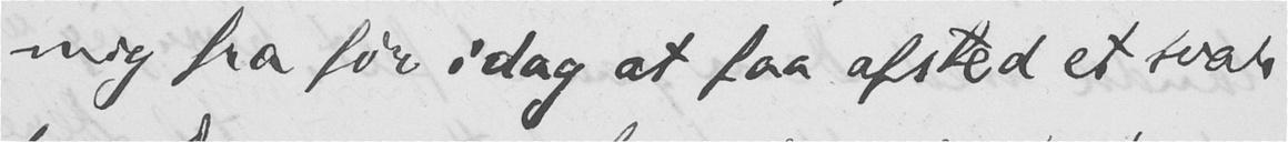mig fra før i dag at faa afsted et svar
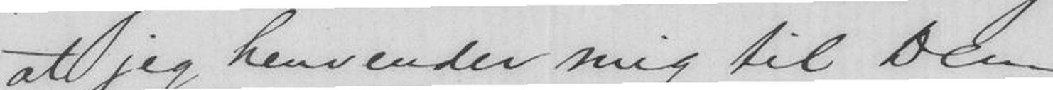at jeg henvender mig til Dem
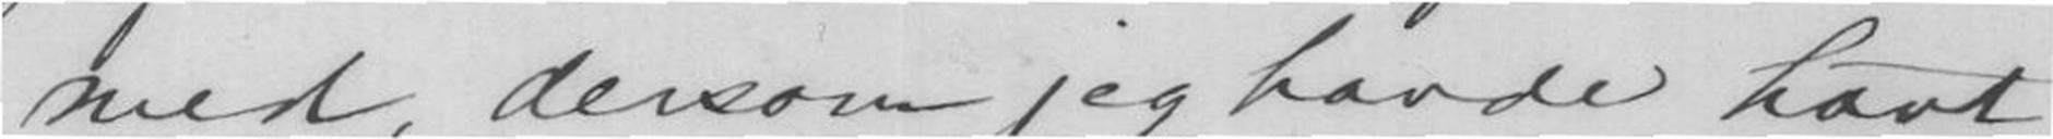med, dersom jeg havde havt
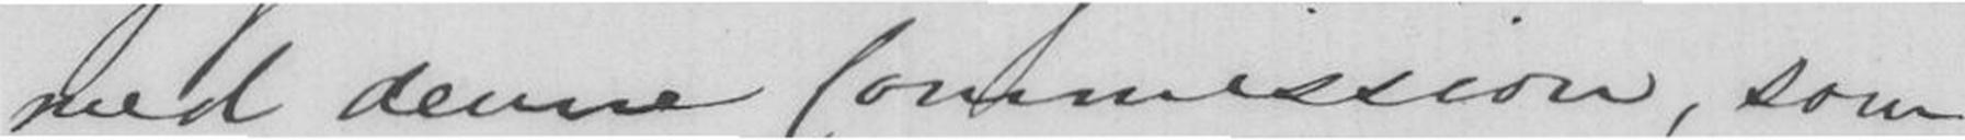med denne Commission, som
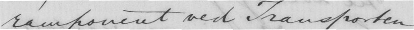ramponeret ved Transporten
